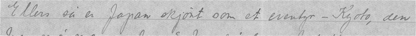Ellers så er Japan skjønt som et eventyr - Kyoto, den
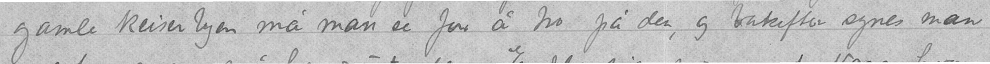gamle keiserbyen må man se for å tro på den, og bakefter synes man
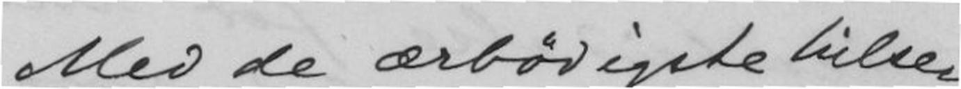Med de ærbødigste hilse
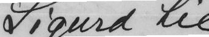Sigurd Lie
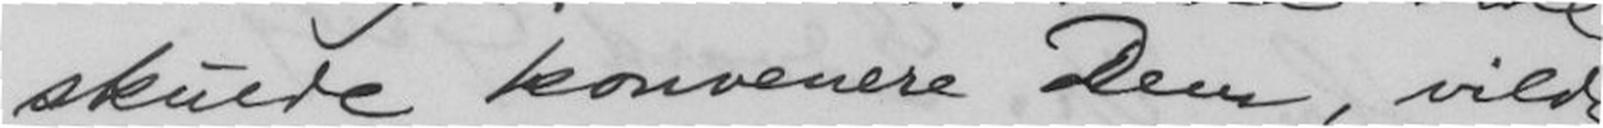skulde konvenere Dem, vilde
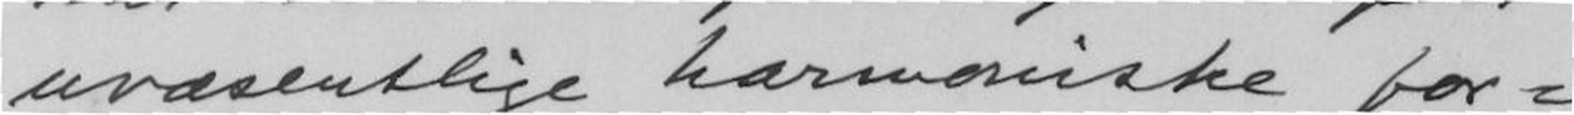uvæsentlige harmoniske for-
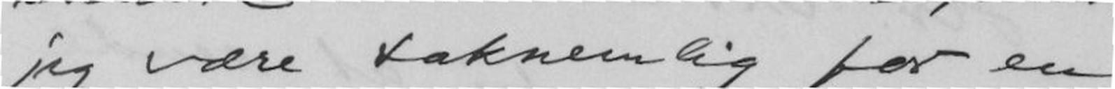jeg være taknemlig for en
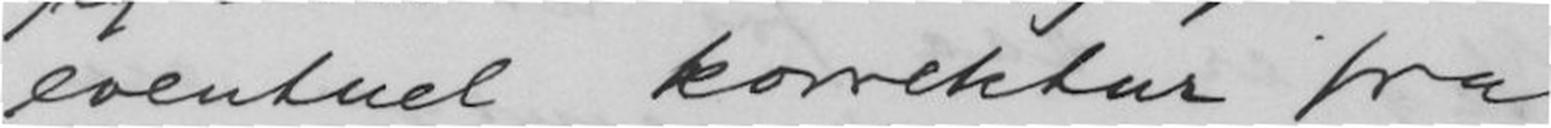eventuel korrektur fra
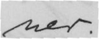ner.
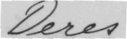Deres
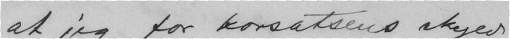at jeg for korsatsens skyld
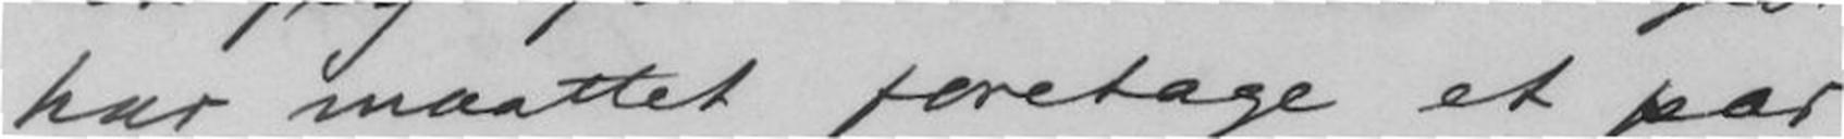har maattet foretage et par
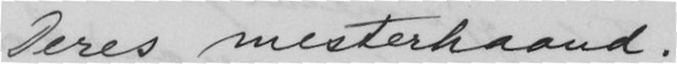Deres mesterhaand.
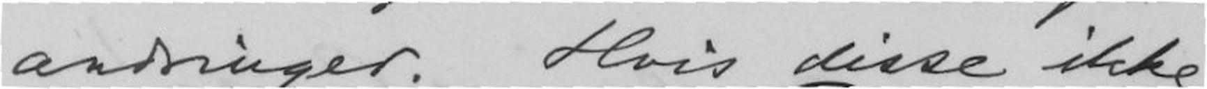andringer. Hvis disse ikke
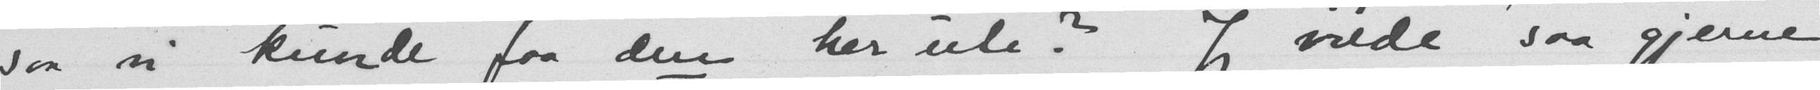saa vi kunde faa den her ute? Jeg vilde saa gjerne
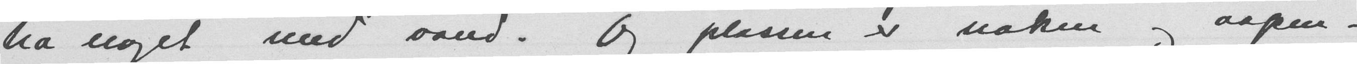ha noget med vand. Og plassen er naken og aapen -
